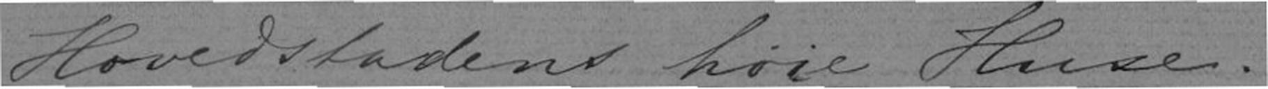Hovedstadens høie Huse.
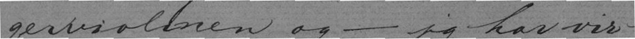gerviolinen og - jeg har vir-
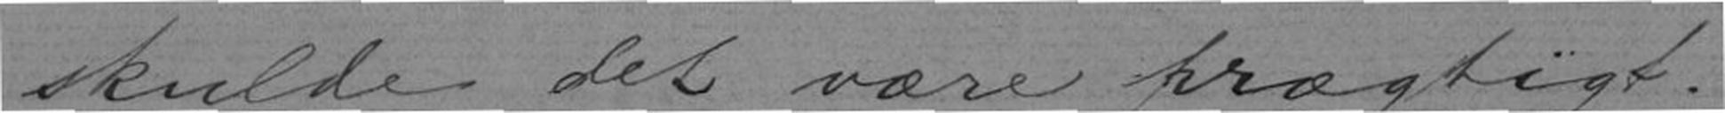skulde det være prægtigt.
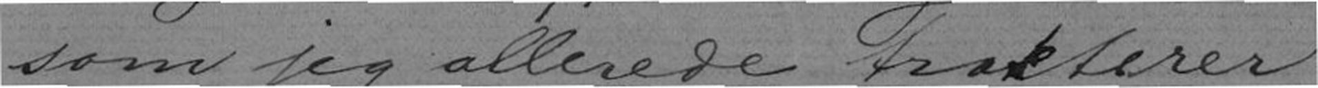som jeg allerede trakterer
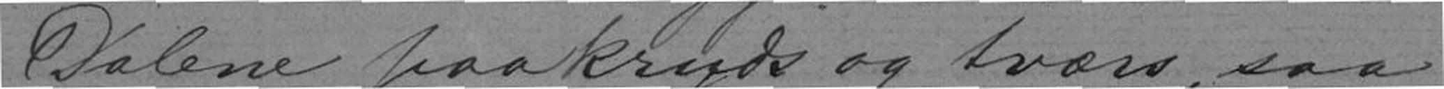Dalene paakryds og tværs, saa
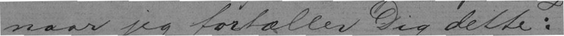naar jeg fortæller Dig dette:
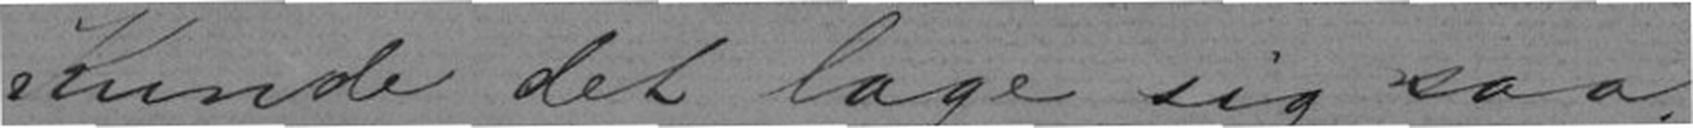Kunde det lage sig saa
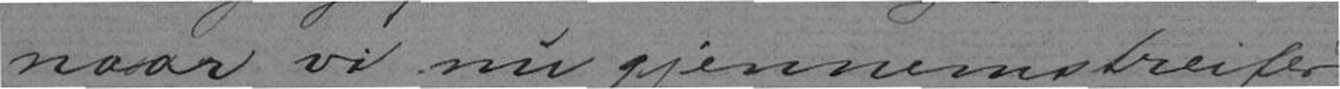naar vi nu gjennemstreifer
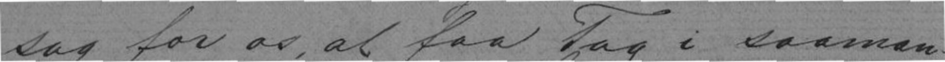sag for os, at faa Tag i saaman-
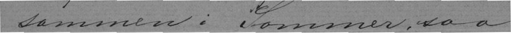sammen i Sommer, saa
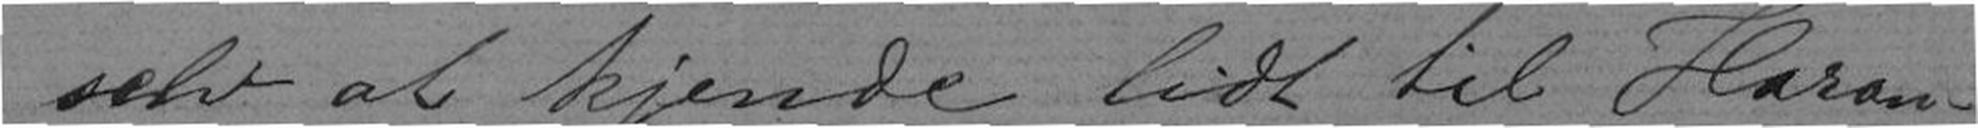selv at kjende lidt til Haran-
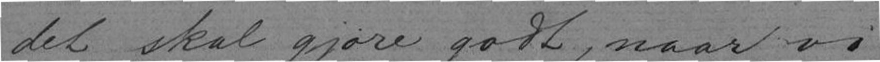det skal gjøre godt, naar vi
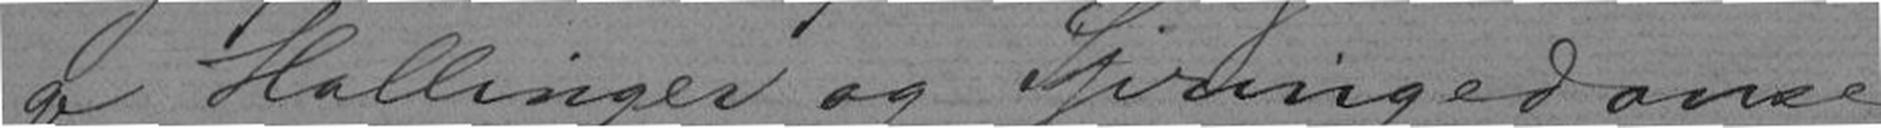ge Hallinger og Springedanse
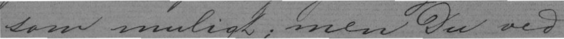som muligt; men Du ved
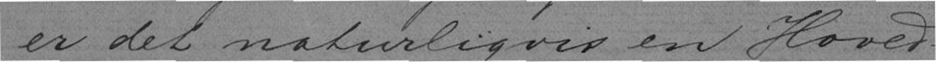er det naturligvis en Hoved-
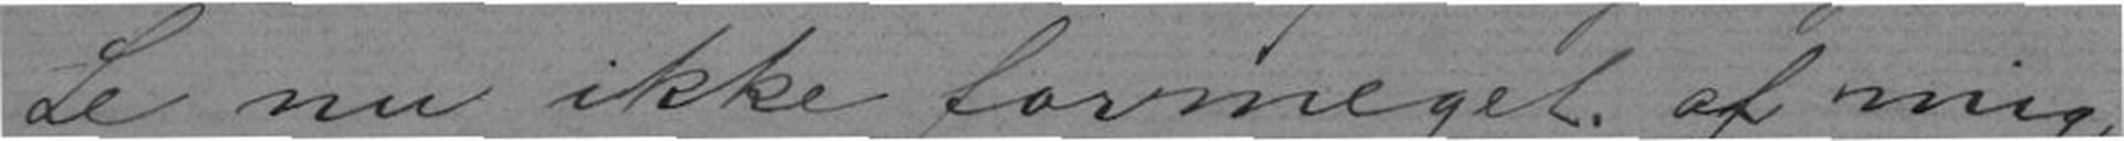Le nu ikke formeget af mig
